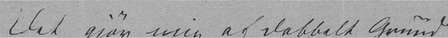Det gjør mig af dobbelt Grund
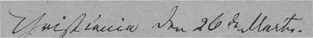Christiania den 26de Marts.
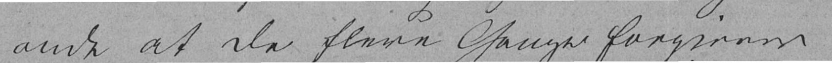ondt at De flere Gange forgjeves
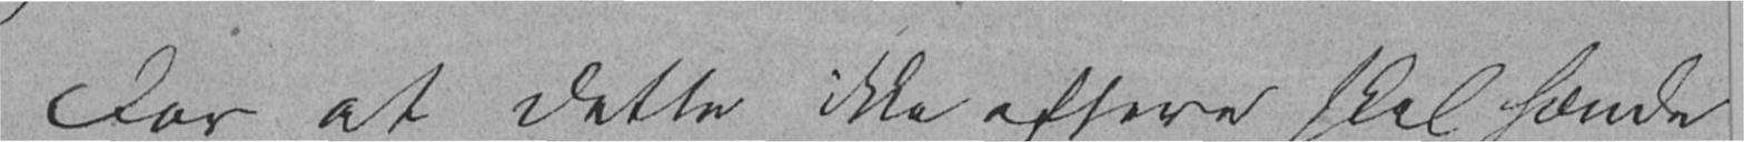For at dette ikke oftere skal hænde
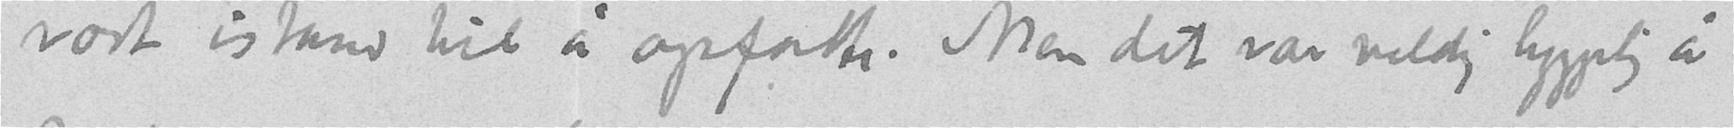vært istand til å opfatte. Men det var veldig hyggelig å
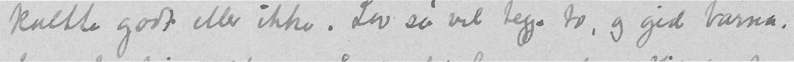kaltte godt eller ikke. Lev så vel begge to, og gid barna,
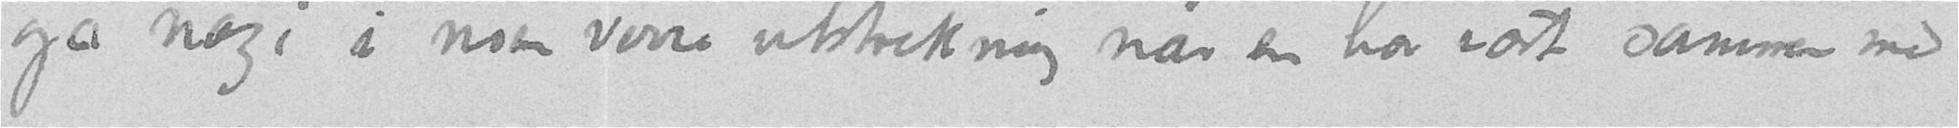gå nazi i noen verre utstrekning når en har vært sammen med
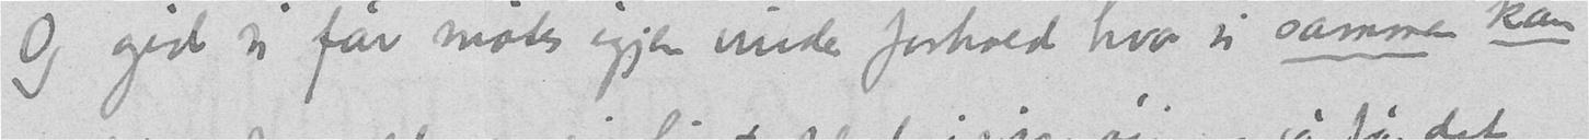Og gid vi får møtes igjen under forhold hvor vi sammen kan
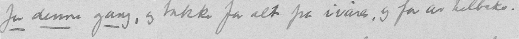for denne gang, og takke for alt fra ivåres, og for år tilbake.
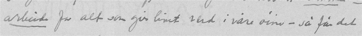arbeide for alt som gir livet verd i våre øine – så får det
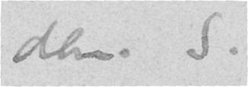dem. S.
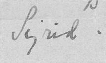Sigrid.
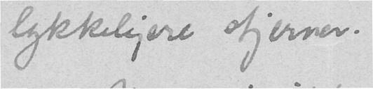lykkeligere stjerner.
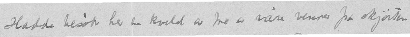Hadde besøk her en kveld av tre av våre venner fra skjørten
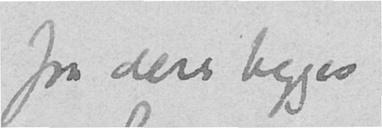fra dere begges
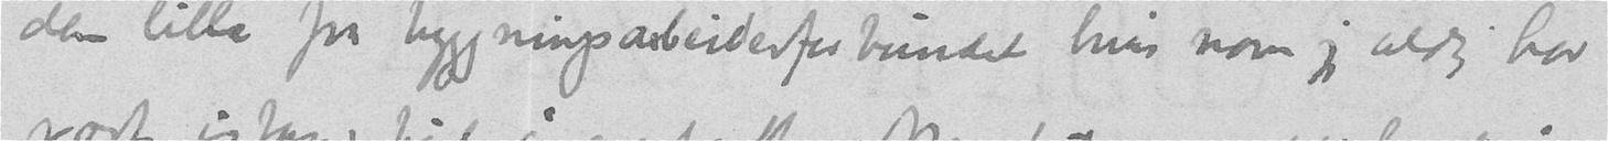den lille fra byggningsarbeiderforbundet hvis navn jeg aldrig har
